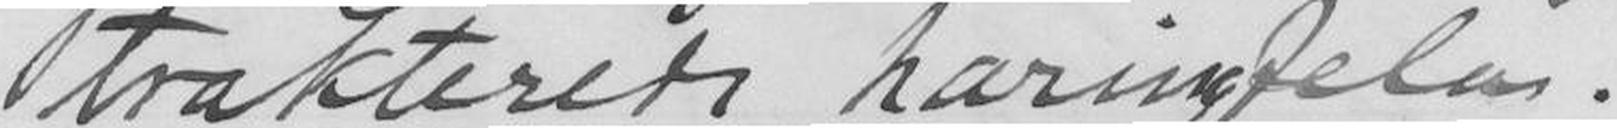trakterede haringfelen.
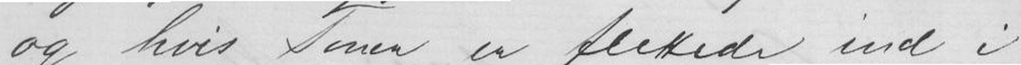og hvis Tonen er flettede ind i
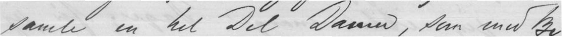samle en hel Del Damer, som med Be-
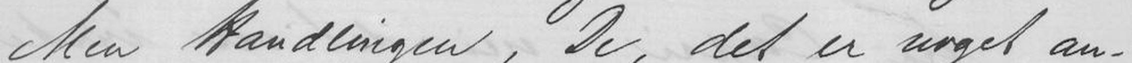Men Handlingen i De, det er noget an-
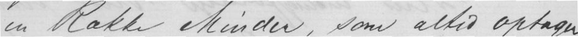en Række Minder, som altid optager
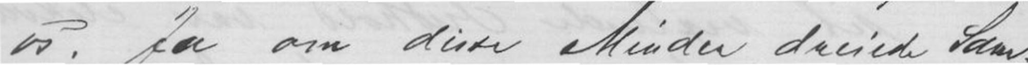os for om disse Minder dreiede Sam-
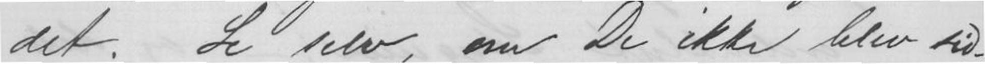det. Se selv, om De ikke blev sid-
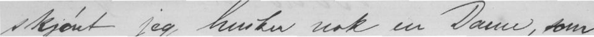skjønt jeg husker nok en Dame, som
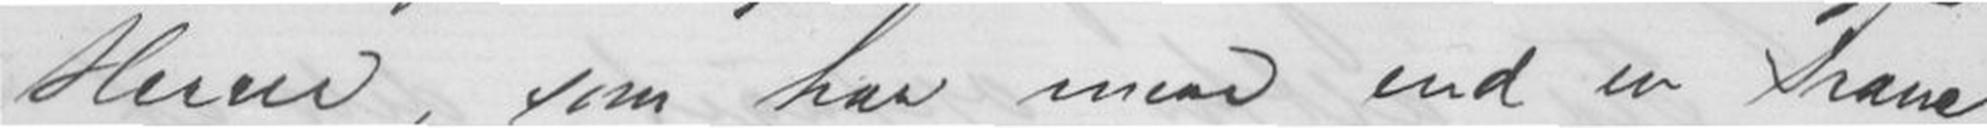Herrer, som har mere end en Franc
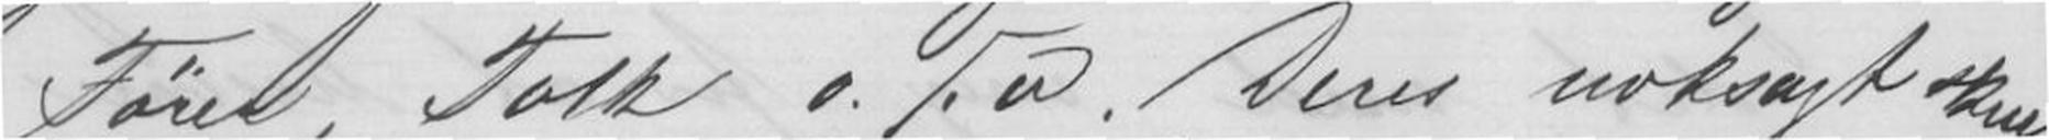Fører, Folk o.s.v. Deres noksagt skul-
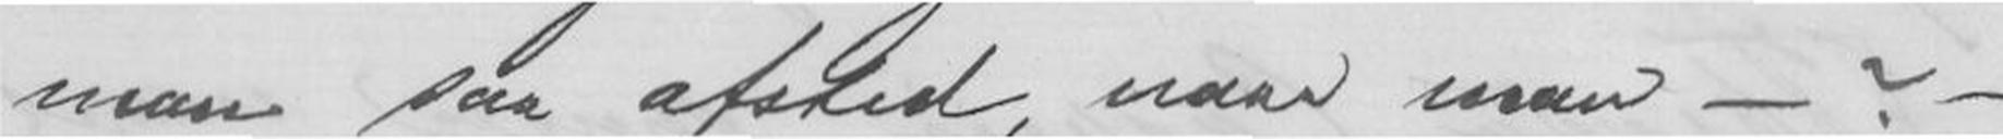man saa afsted, naar man -?-
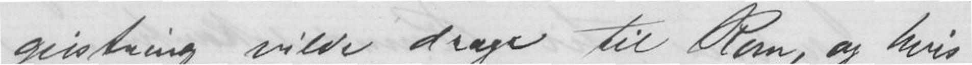geistring vilde drage til Rom, og hvis
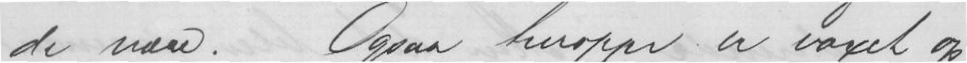de være. Ogsaa heroppe er voxet op
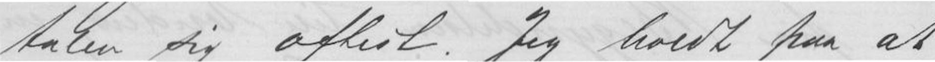talen sig oftest. Jeg holdt paa at
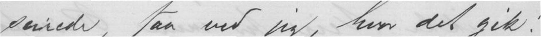svinder, saa ved jeg, hvor det gik!
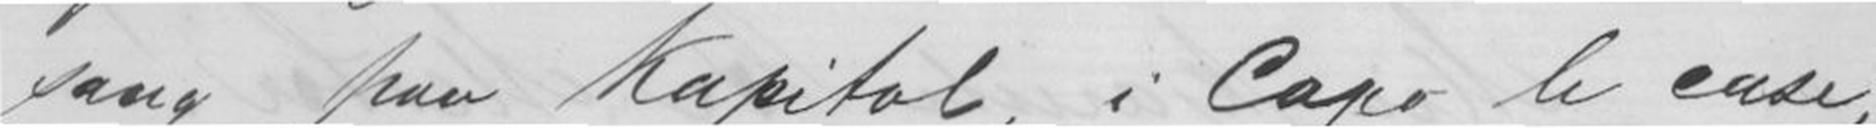sang paa Kapitol, i Capo le ease,
